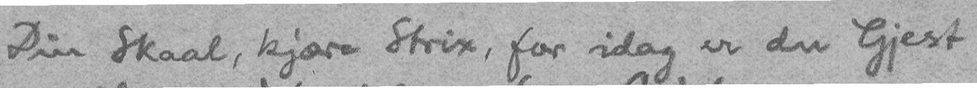Din Skaal, kjære Strix, for idag er du Gjest
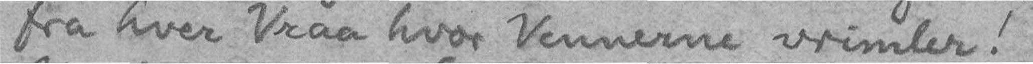fra hver Vraa hvor Vennerne vrimler!
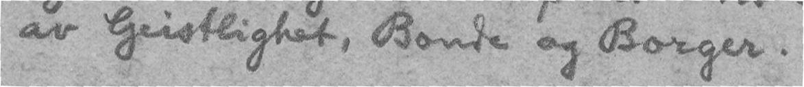av Geistlighet, Bonde og Borger.
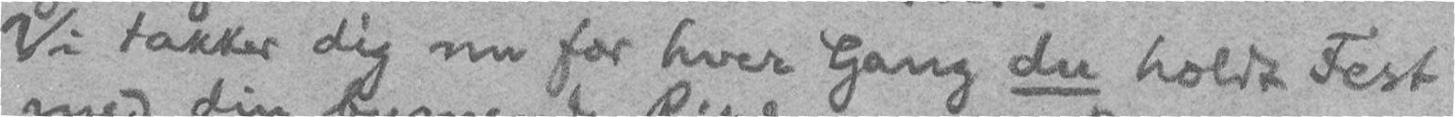Vi takker dig nu for hver Gang du holdt Fest
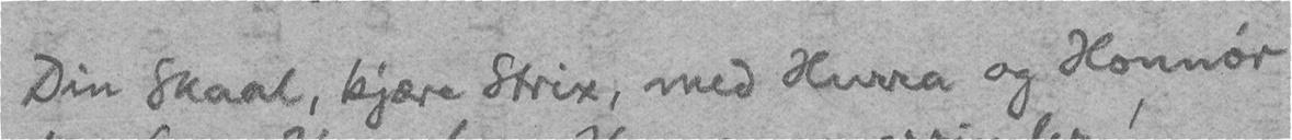Din Skaal, kjære Strix, med Hurra og Honnør
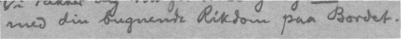med din bugnende Rikdom paa Bordet.
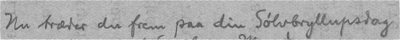Nu træder du frem paa din Sølvbryllupsdag
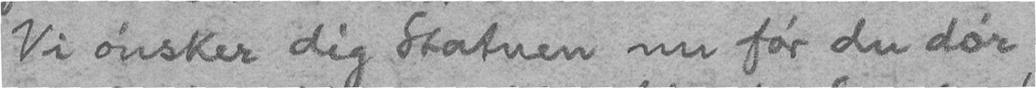Vi ønsker dig Statuen nu før du dør
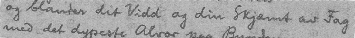og blander dit Vidd og din Skjæmt av Fag
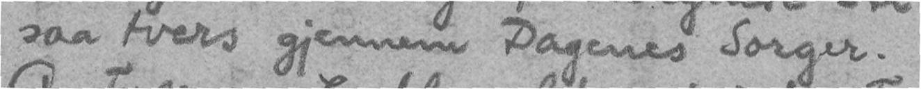saa tvers gjennem Dagenes Sorger.
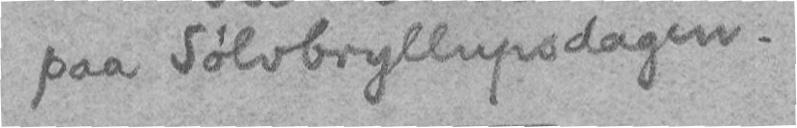paa Sølvbryllupsdagen.
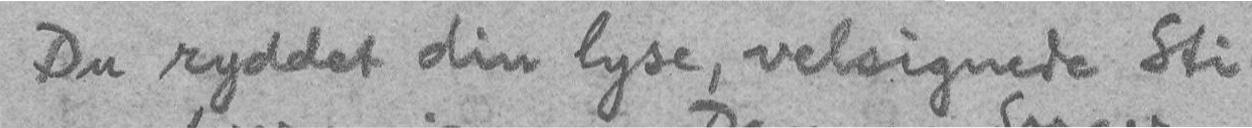Du ryddet din lyse, velsignede Sti
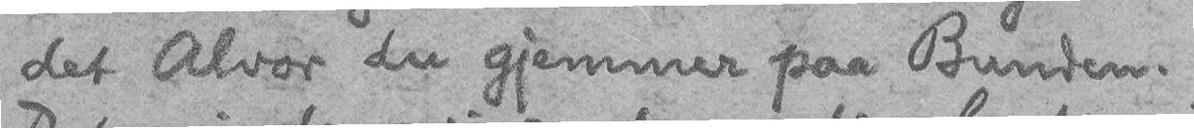det Alvor du gjemmer paa Bunden.
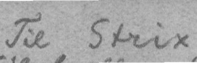Til Strix
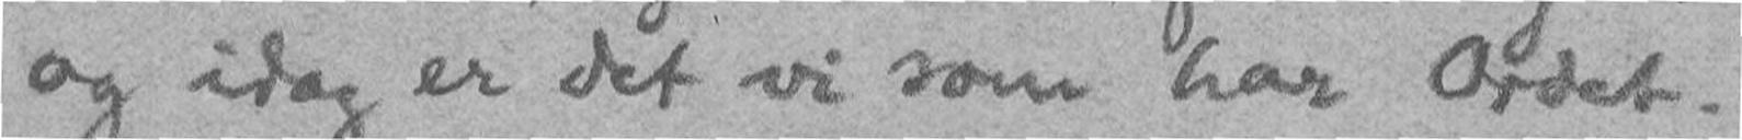og idag er det vi som har Ordet.
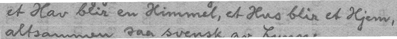et Hav blir en Himmel, et Hus blir et Hjem,
